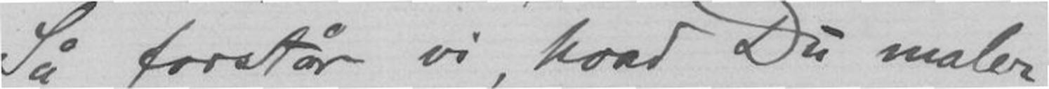Så forstår vi, hvad Du maler,
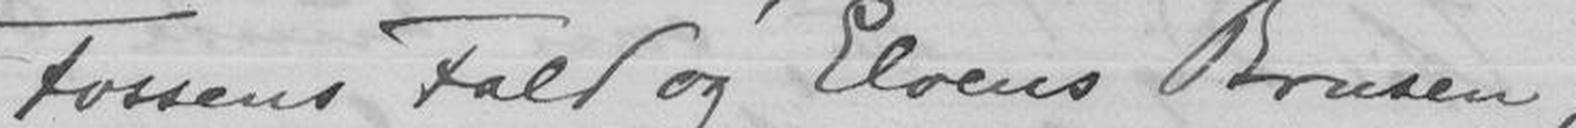Fossens Fald og Elvens Brusen,
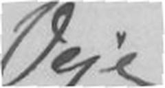Veje,
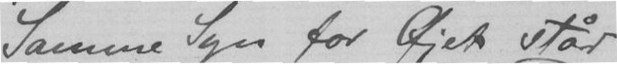Samme Syn for Øjet står.
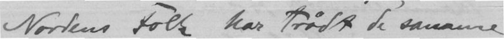Nordens Folk har trådt de samme
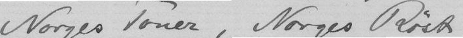Norges Toner, Norges Røst
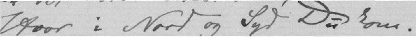Hvor i Nord og Syd Du kom.
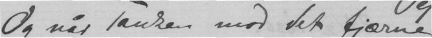Og når Tanken mod det fjerne
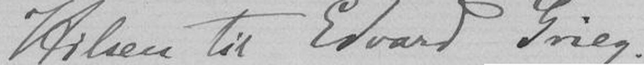Hilsen til Edvard Grieg.
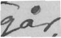går,
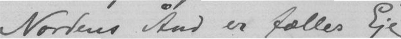Nordens Ånd er fælles Eje,
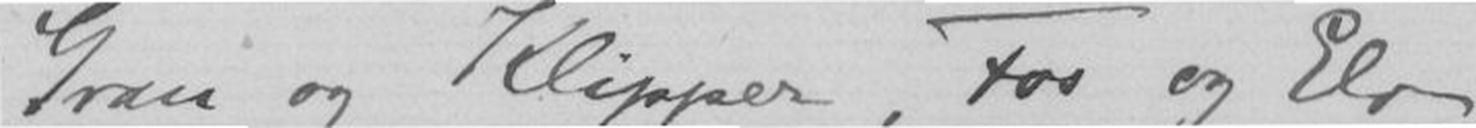Gran og klipper, Fos og Elv
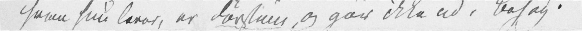hvem hun lever, er døvstum, og går ikke ud i Besøg.
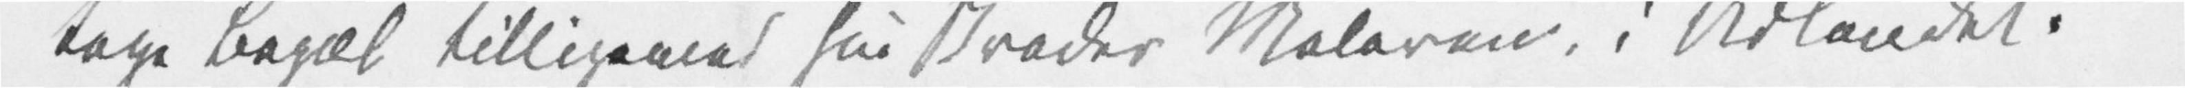tage Bopæl tilligemed sin Broder Maleren, i Udlandet.
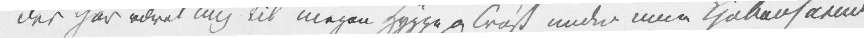der har været mig til megen Hygge og Trøst under mine Kjøbenhavner=
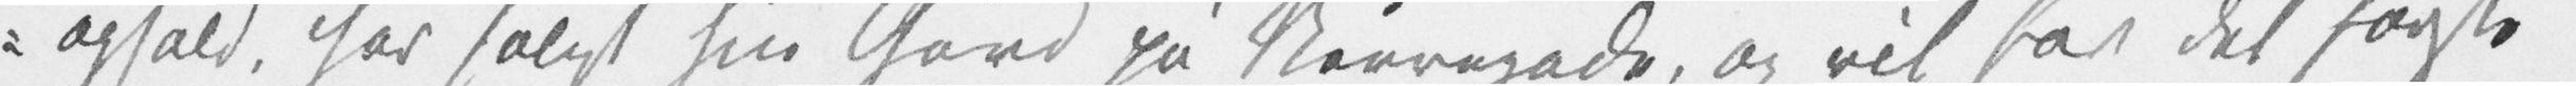ophold, har solgt sin Gård på Nørregade, og vil for det første
